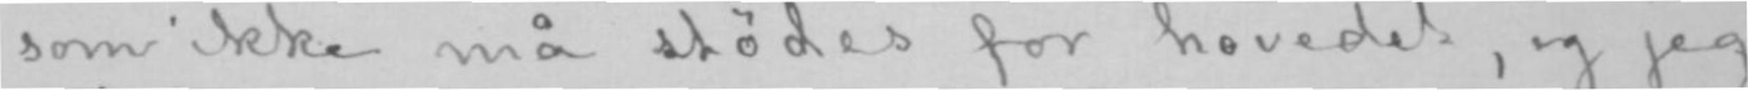som ikke må stødes for hovedet, og jeg
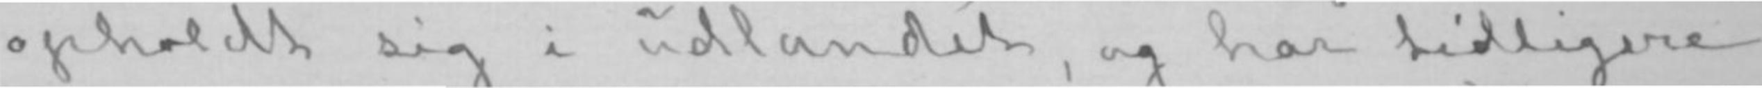opholdt sig i udlandet, og har tidligere
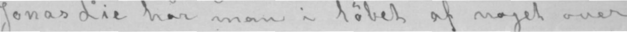Jonas Lie har man i løbet af noget over
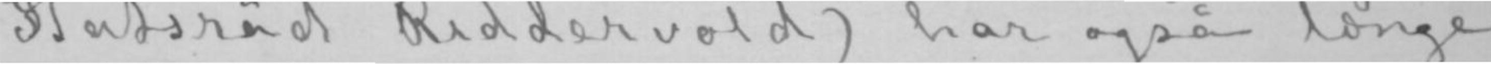Sstatsråd Riddervold) har også længe
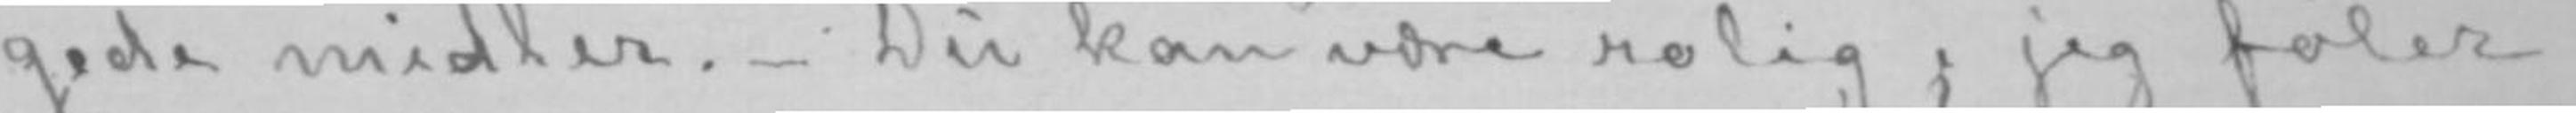gede midler.- Du kan være rolig; jeg føler
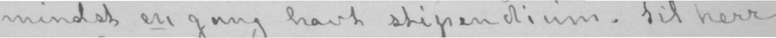mindst en gang havt stipendium. Til herr
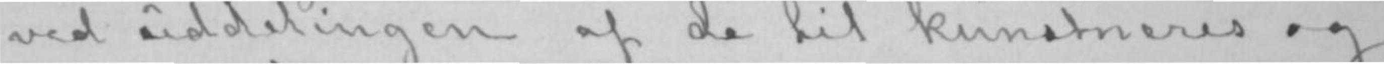ved uddelingen af de til kunstneres og
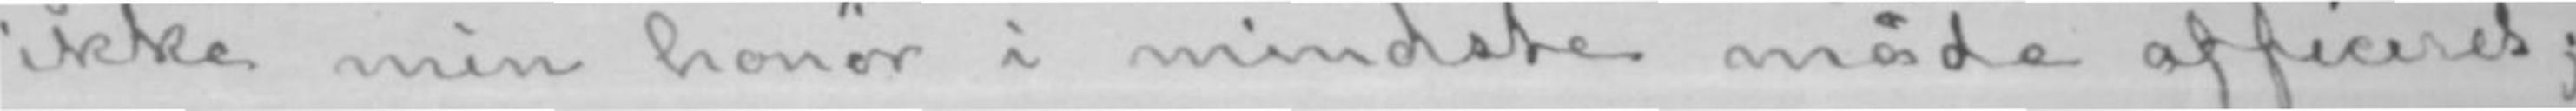ikke min honør i mindste måde afficeret;
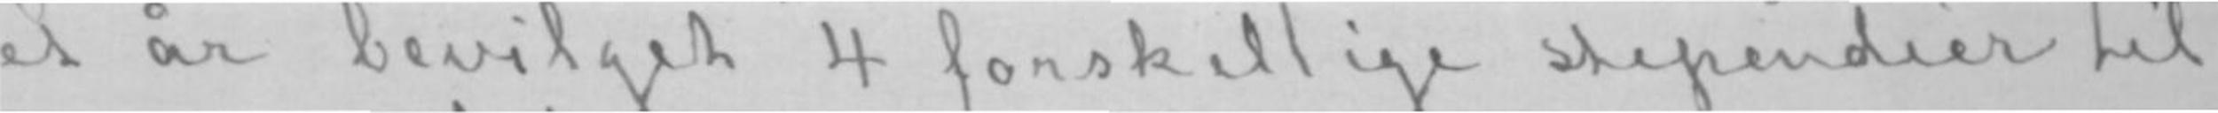et år bevilget 4 forskellige stipendier til
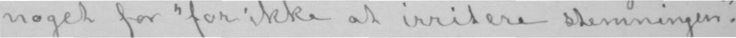noget for «for ikke at irritere stemningen».
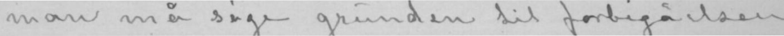man må søge grunden til forbigåelsen
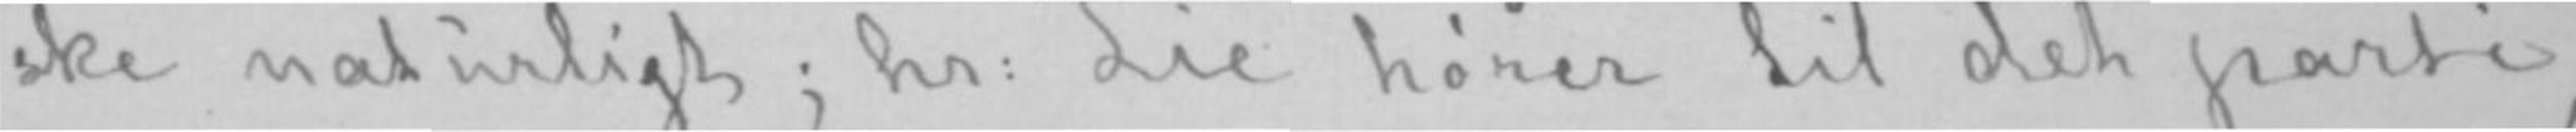ske naturligt; hr: Lie hører til det parti,
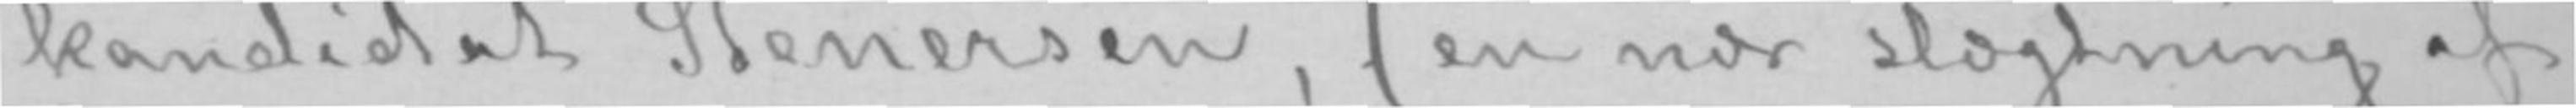kandidat Stenersen, (en nær slægtning af
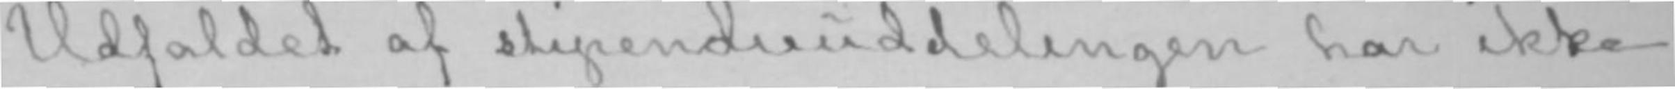Udfaldet af stipendieuddelingen har ikke
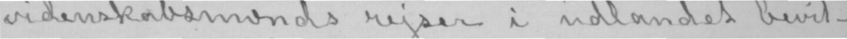videnskabsmænds rejser i udlandet bevil-
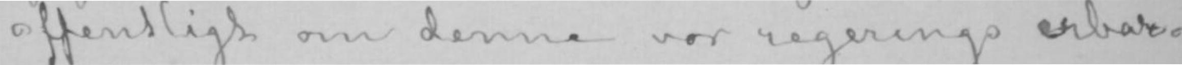offentligt om denne vor regerings erbar-
